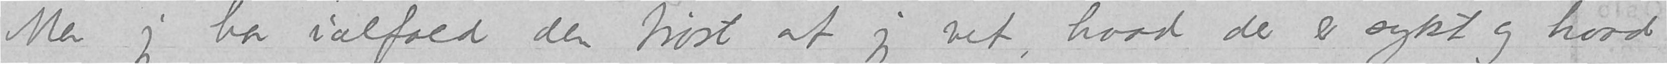Men jeg har ialfald den trøst at jeg vet, hvad der er sykt og hvad
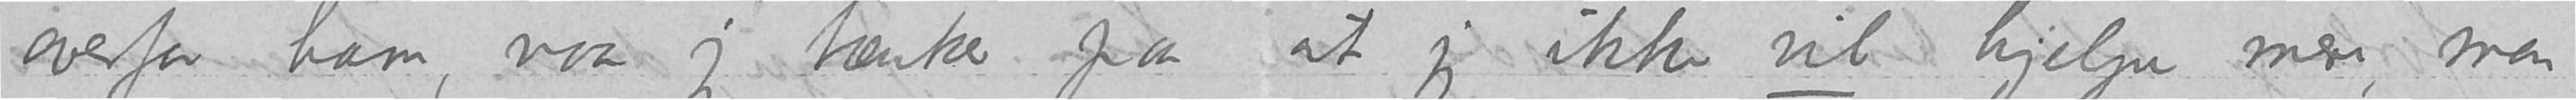overfor ham, naar jeg tænker paa at jeg ikke vil hjelpe mere, men jeg
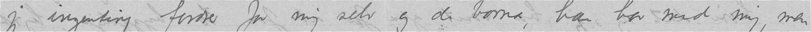jeg ingenting fordrer for mig selv og de barna, han har med mig, men
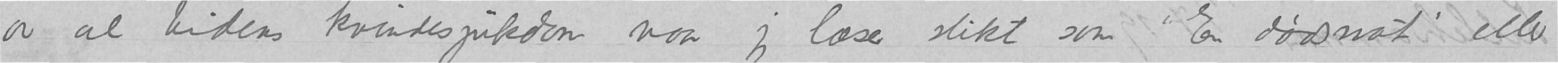av al tidens kvindesjukdom naar jeg læser slikt som «En dødsnat» eller
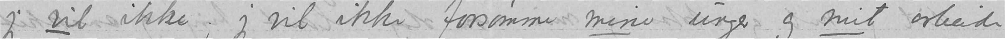vil ikke; jeg vil ikke forsømme mine unger og mit arbeide
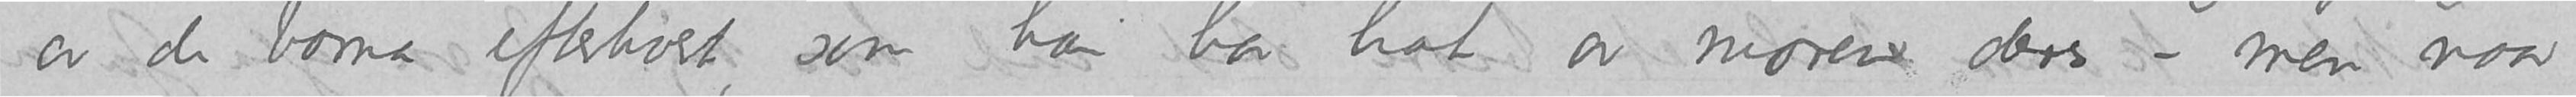av de barna efterhvert, som han har hat av moren deres – men naar
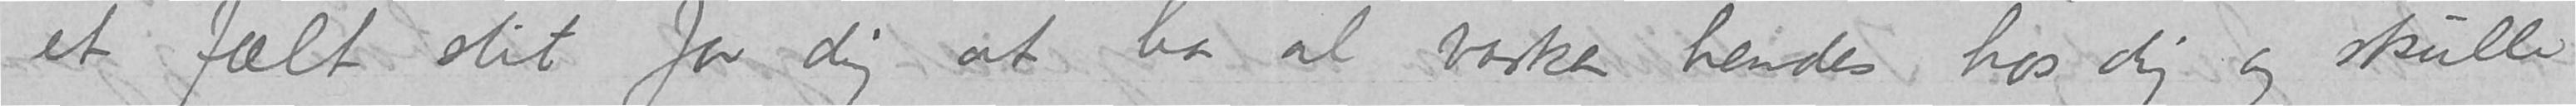et fælt slit for dig at ha al  vasken hendes hos dig og skulle
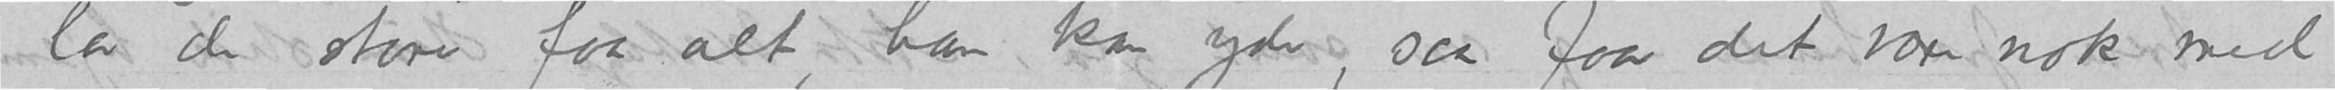lar de store faa alt, han kan yde, saa faar det være nok med
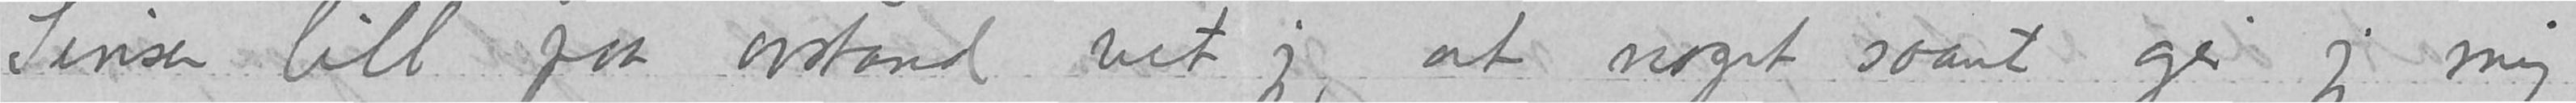avstand vet jeg, at noget saant gir jeg mig
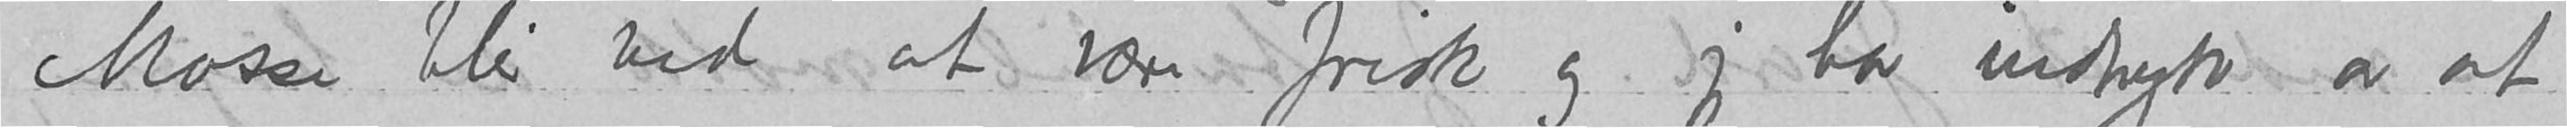Mosse blir ved at være frisk og jeg har indtryk av at
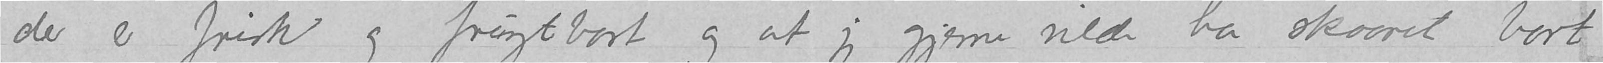der er friskt og frugtbart og at jeg gjerne vilde ha skaaret bort
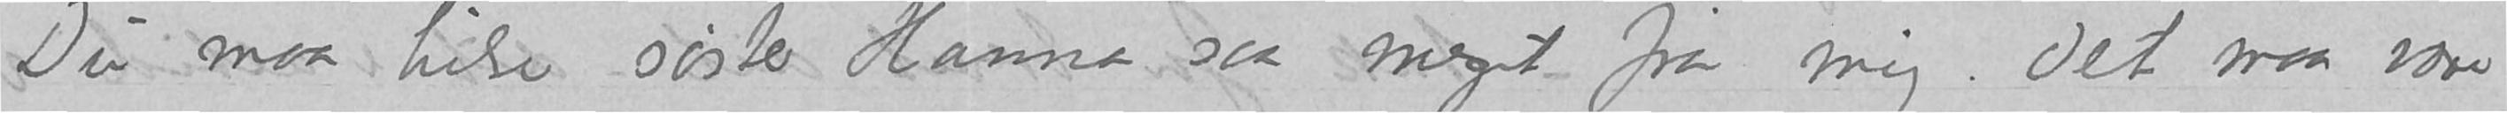Du maa hilse søster Hanna saa meget fra mig. Det maa være
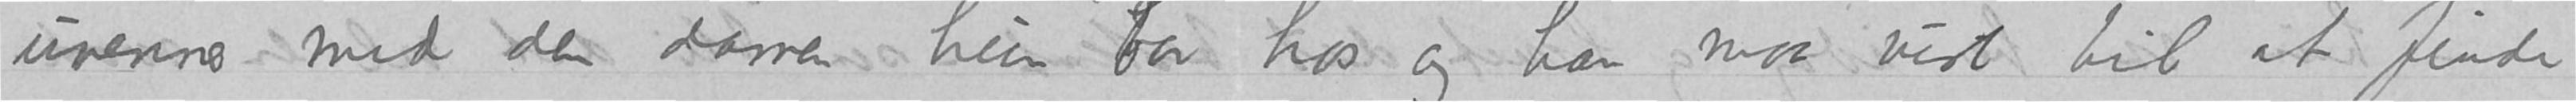uvenner med den damen hun bor hos og han maa vist til at finde
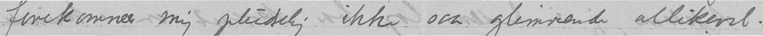og blod forekommer mig pludselig ikke saa glimrende allikevel.
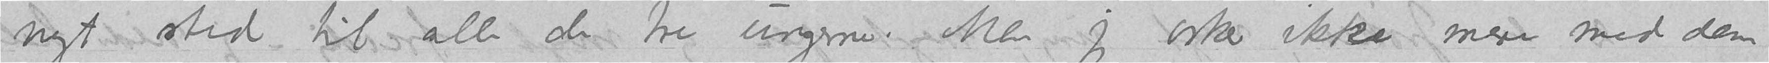nyt sted til alle de tre ungerne. Men jeg orker ikke mere med dem
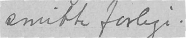smittefarlige.
